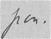paa.
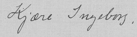Kjære Ingeborg,
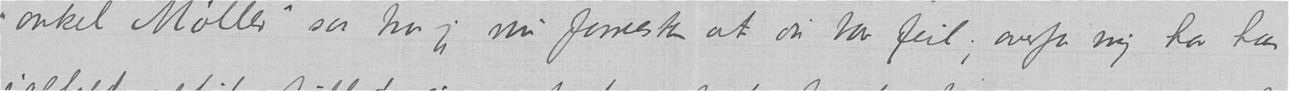"onkel Møller" saa tror jeg nu forresten at du tar feil; overfor mig har han
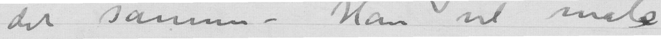det samme – Han vil male
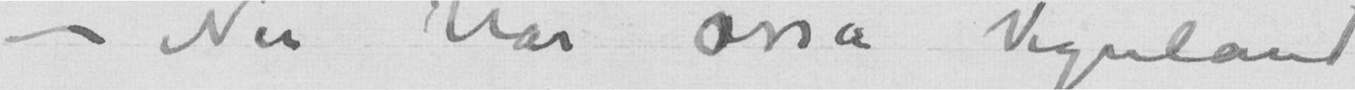– Nu har osså Vigeland
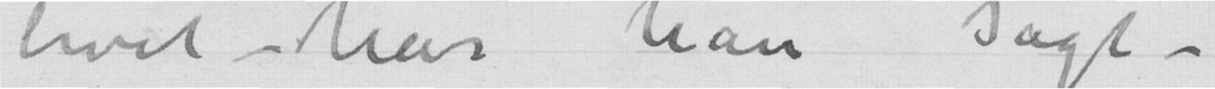livet – har han sagt –
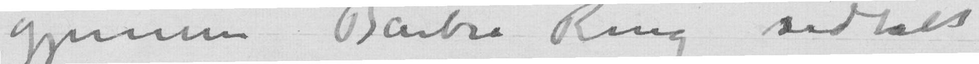gjennem Barbra Ring udtalt
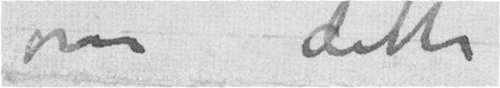om dette
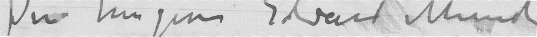Din hengivne Edvard Munch
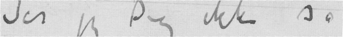Ser jeg Dig ikke så
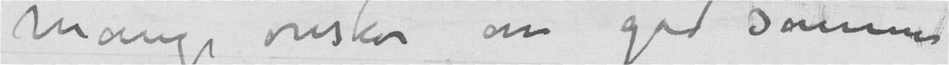mange ønsker om god sommer
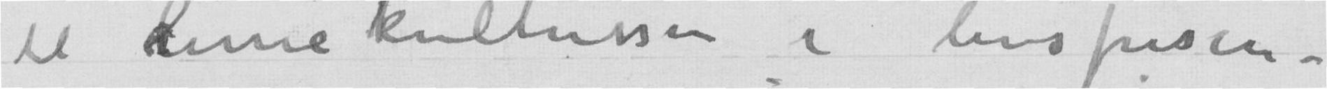til liniekultussen i livsfrisen –
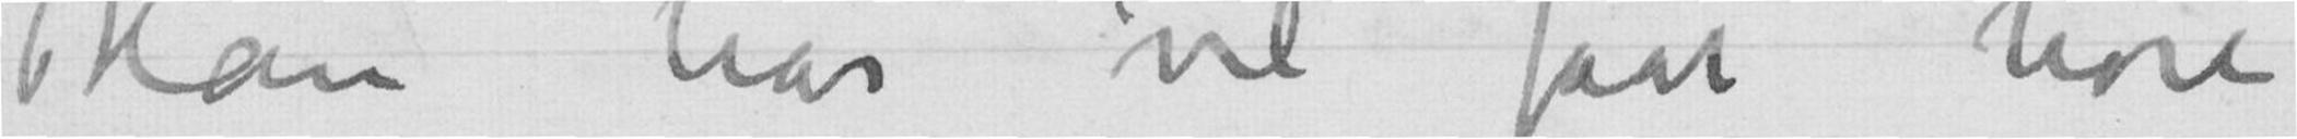Han har vel faat høre
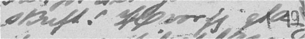skrift. Hvor jeg glæ-
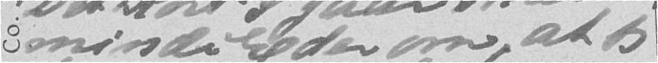minde Eder om, at jeg
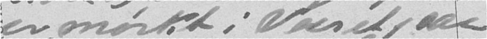er mørkt i Været, saa
